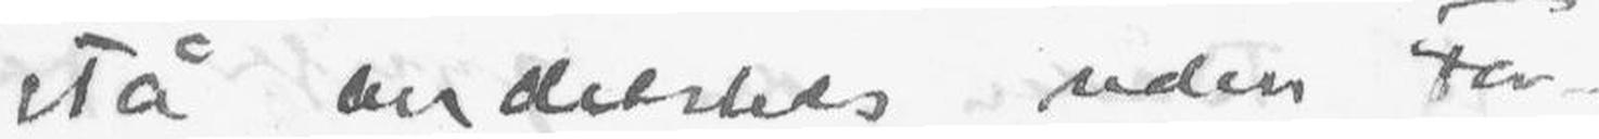stå andetsteds uden For-
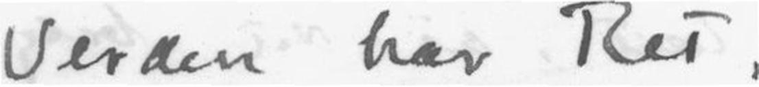Verden har Ret.
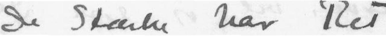De Stærke har Ret.
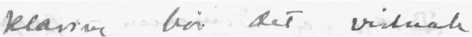klaring bør det vistnok
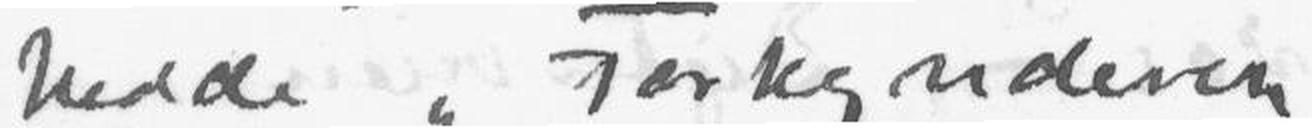hedde Forkynderen
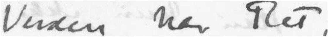Verden har Ret.
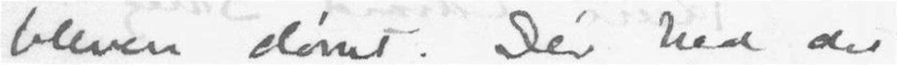bleven dømt. Dèr hed det
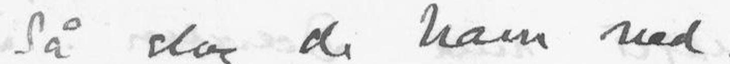Så slog de ham ned.
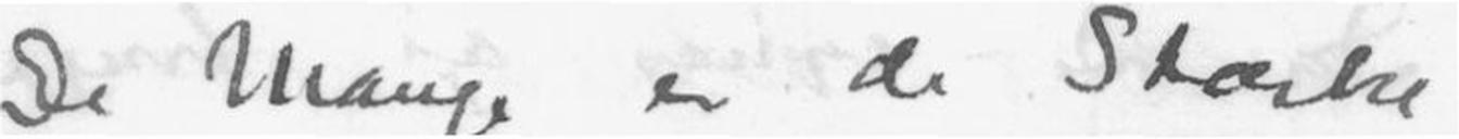De Mange er de Stærke,
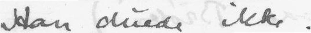Han duede ikke.
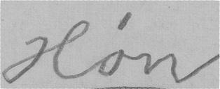Høn
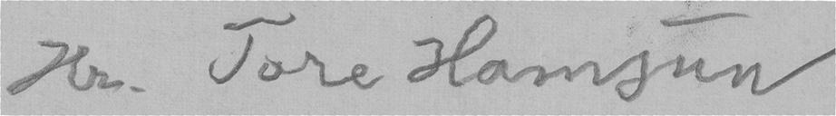Hr. Tore Hamsun
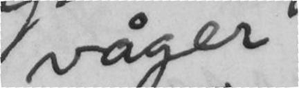våger
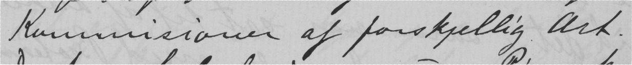Kommisioner af forskjellig Art.
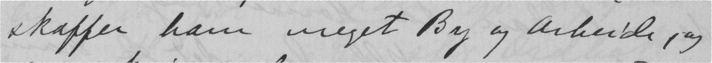skaffer ham meget By og arbeide, og
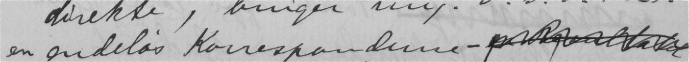en endeløs Korrespandense -
